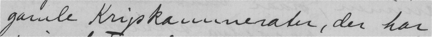gamle Krigskammerater, der har
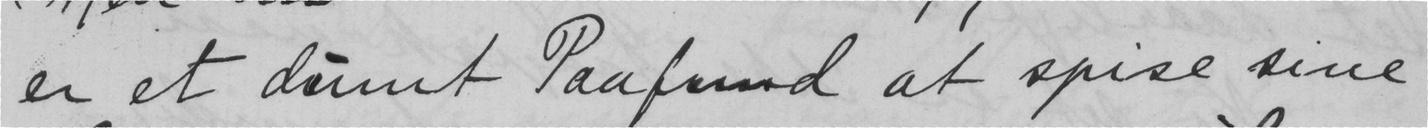er et dumt Paafund at spise sine
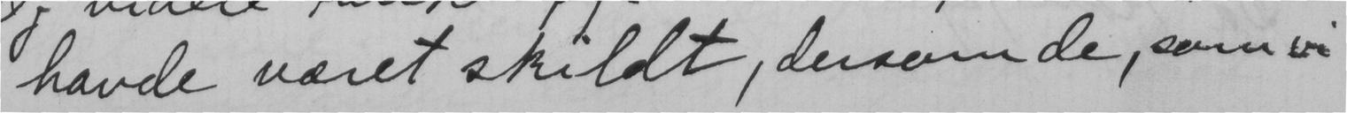havde været skildt, dersom de, som vi
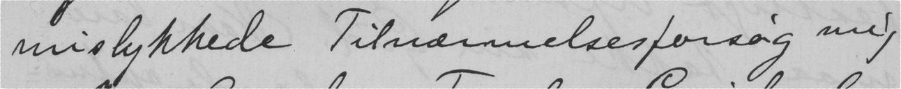mislykkede Tilnærmelsesforsøg mig
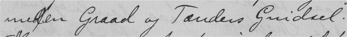megen Graad og Tænders Gnidsel.
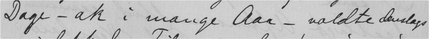Dage - ak i mange Aar - voldte denslags
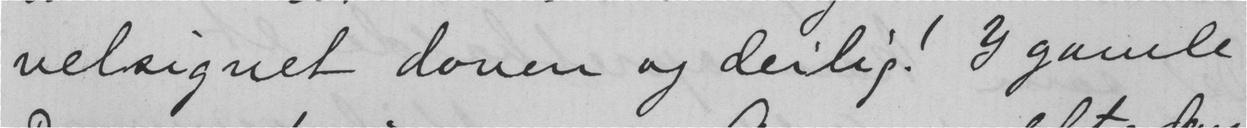velsignet doven og deilig! I gamle
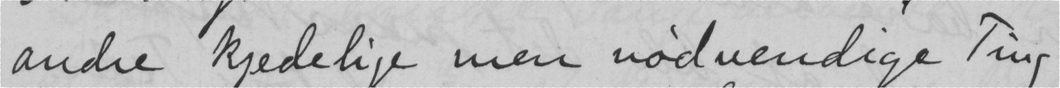andre kjedelige men nødvendige Ting
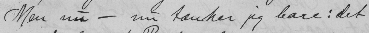Men nu - nu tænker jeg bare: det
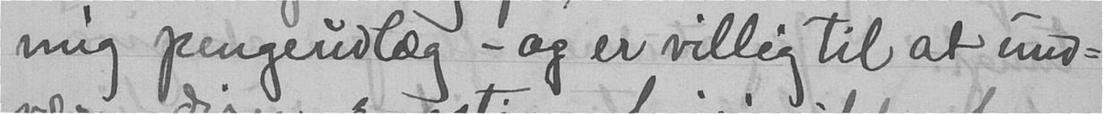mig pengeudlæg - og er villig til at und-
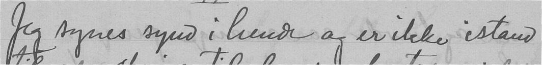Jeg synes synd i hende og er ikke istand
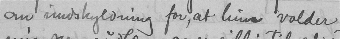om undskyldning for, at hun volder
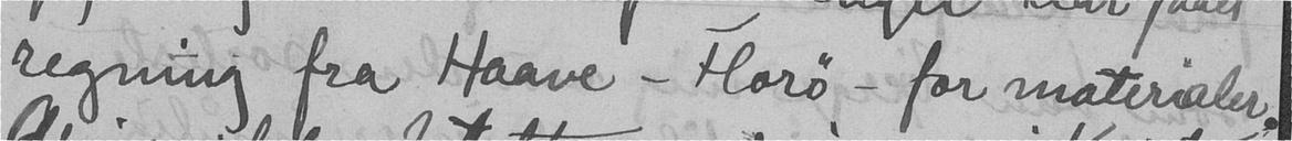regning fra Haave - Horø - for materialer.
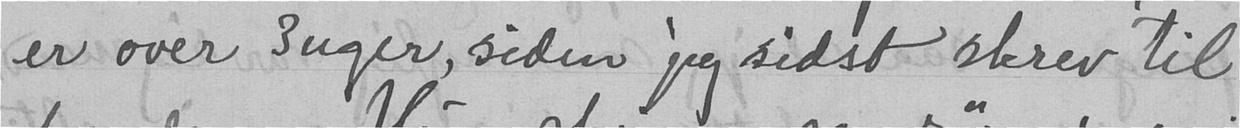er over 3 uger, siden jeg sidst skrev til
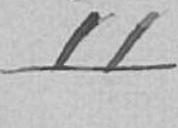II
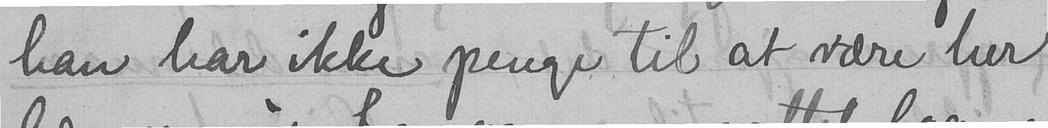han har ikke penge til at være her
